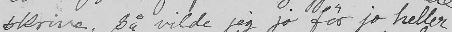skrive, så vilde jeg jo før jo heller
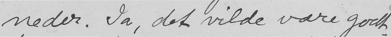neder. Ja, det vilde være godt.
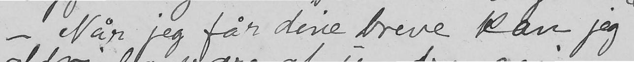- Når jeg får dine breve kan jeg
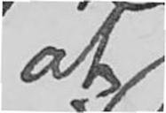at
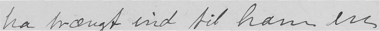ha trængt ind til ham en
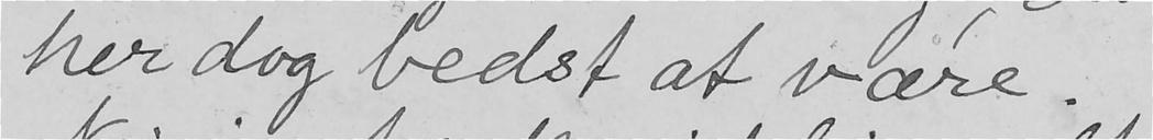her dog bedst at være.
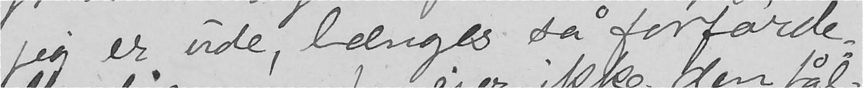jeg er ude, længes så forfærde-
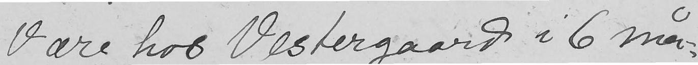Være hos Vestergaard i 6 må-
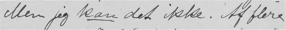eller jeg kan det ikke. Af flere
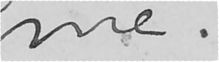me.
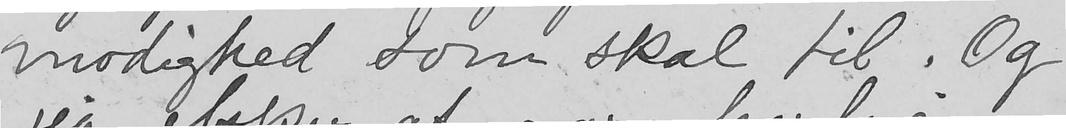modighed som skal til. Og
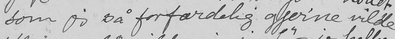som jeg så forfærdelig gjerne vilde
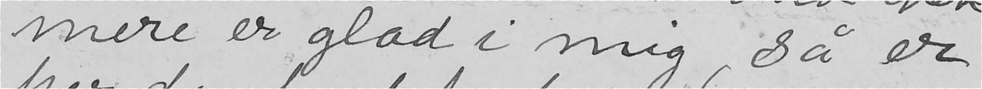mere er glad i mig, så er
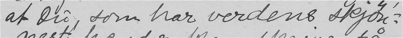at Du, som har verdene skjøn-
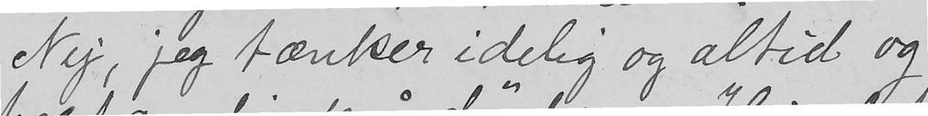Nej, jeg tænker idelig og altid og
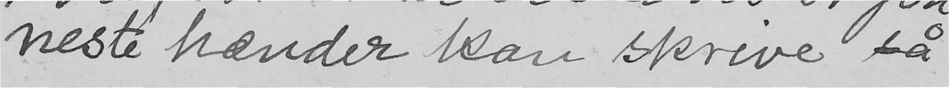neste hænder kan skrive så
